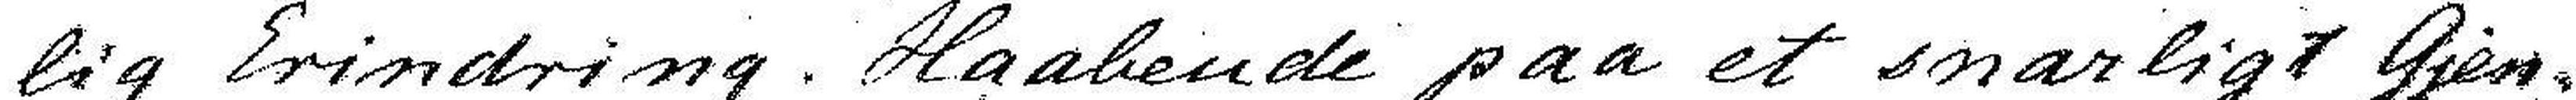lig Erindring. Haabende paa et snarligt Gjen-
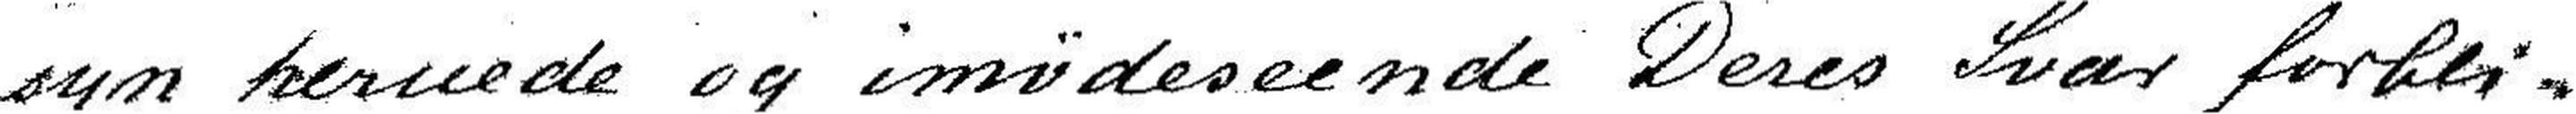syn hernede og imødeseende Deres Svar forbli-
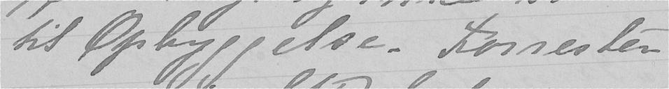til Opbyggelse. Forresten
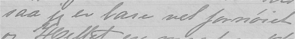saa jeg er bare vel fornøiet
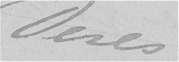Deres
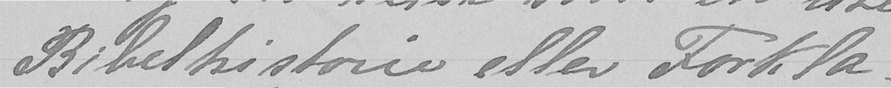Bibelhistorie eller Forkla-
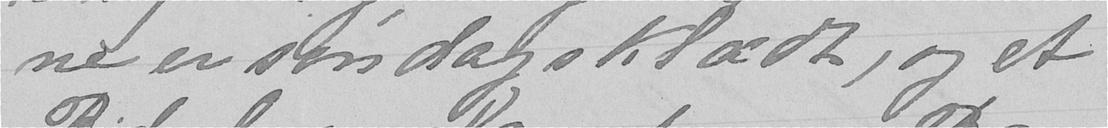ne er søndagsklædt, og et
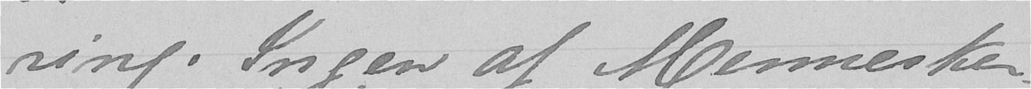ring. Ingen af Mennesker-
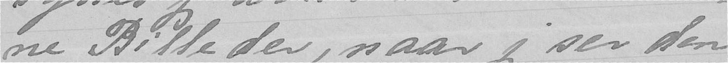ne Billeder, naar jeg ser den
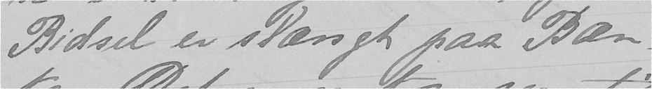Bidsel er slængt paa Bæn-
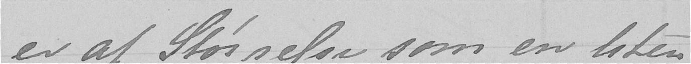er af Størrelse som en liten
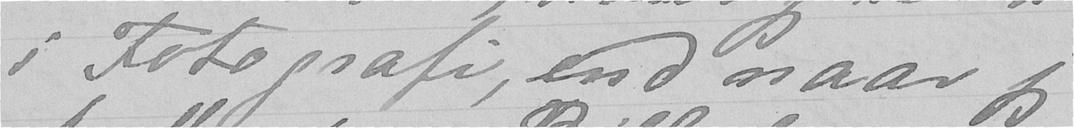i Fotografi, end naar jeg
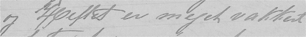og Heftet er meget vakkert
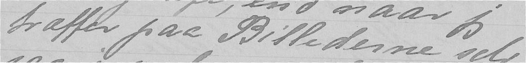træffer paa Billederne selv,
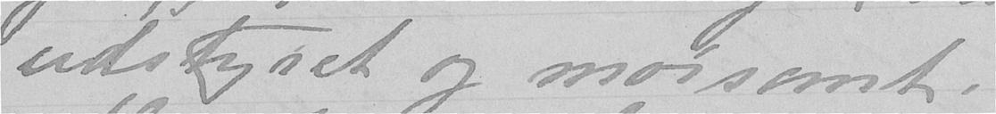udstyret og morsomt.
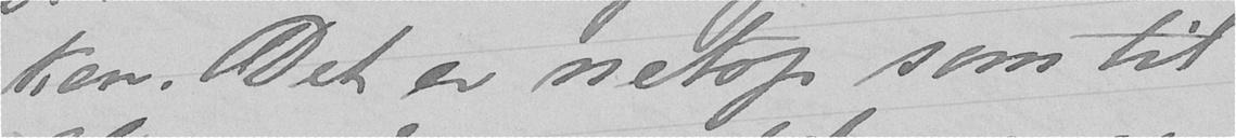ken. Det er netop som til
